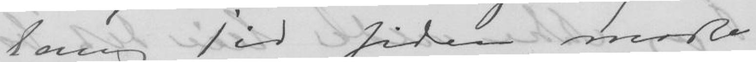lang Tid siden modta-
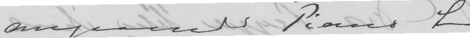angaaende Piano til
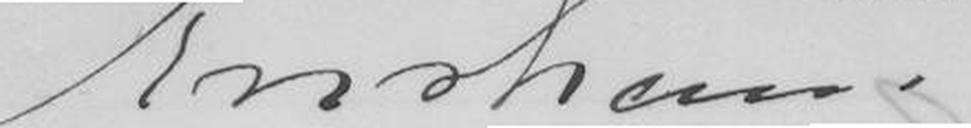Kristiania
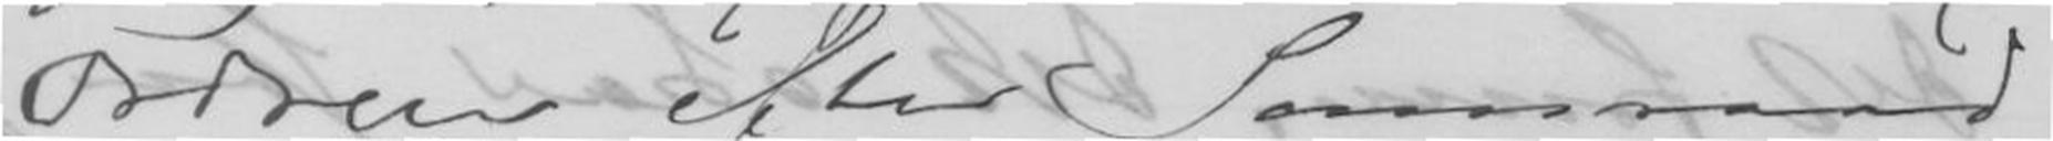Ordren efter Samraad
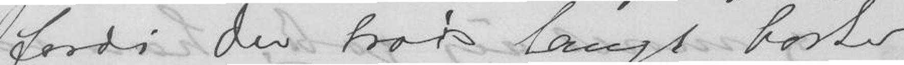fordi du trods langt borte
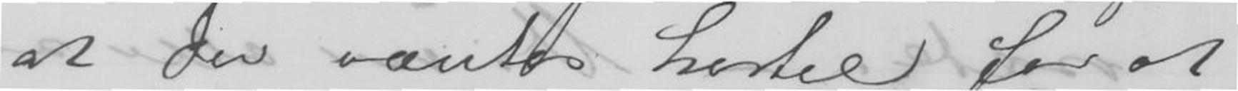at du væntes hertil for at
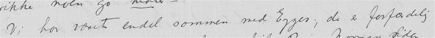Vi har været endel sammen med Egges; de er forfærdelig
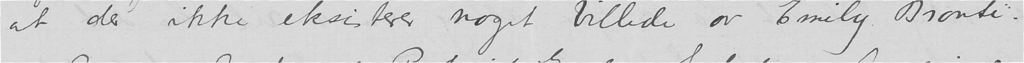at der ikke eksisterer noget billede av Emily Brontë.
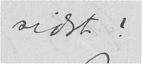sidst?
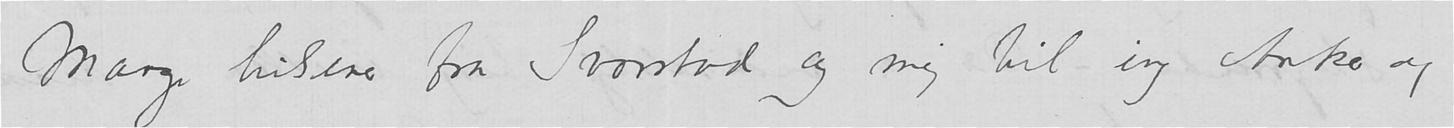Mange hilsener fra Svarstad og mig til ing Anker og
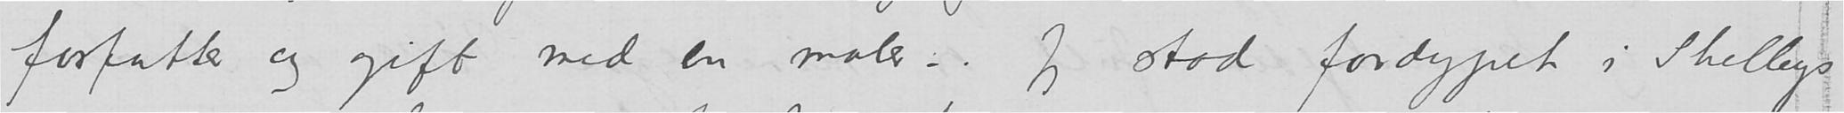forfatter og gift med en maler –. Jeg stod fordypet i Shelleys
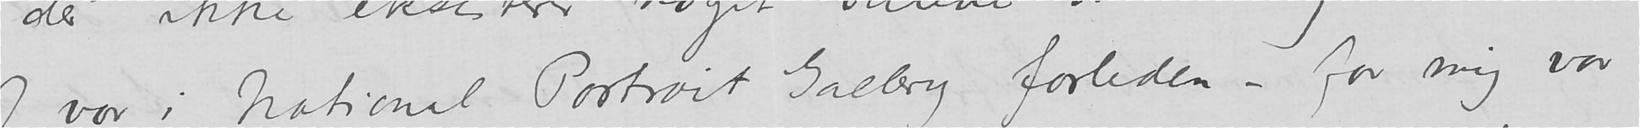– Jeg var i National Portrait Gallery forleden – for mig var
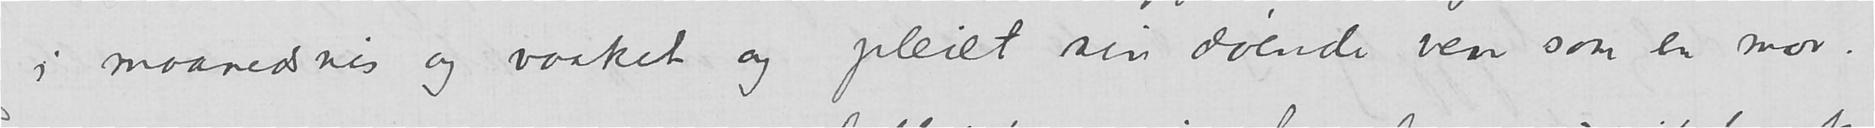i maanedsvis og vaaket og pleiet sin døende ven som en mor.
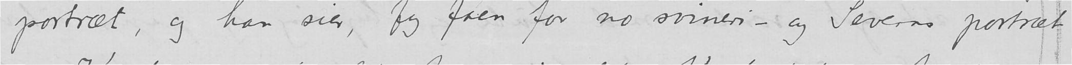portræt, og han sier, fy faen for no svineri – og Severns portræt
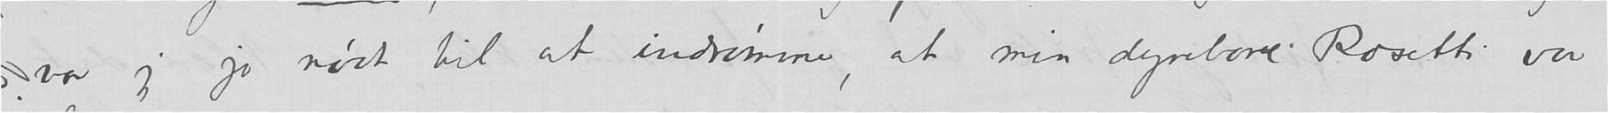var jeg jo nødt til at indrømme, at min dyrebare Rosetti var
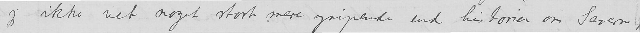jeg ikke vet noget stort mere gripende end historien om Severn,
